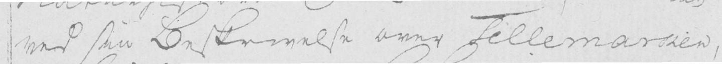ved sin Beskrivelse over Tellemarken,
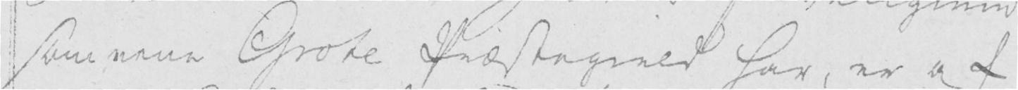som eene Grote Præstegield har, er af
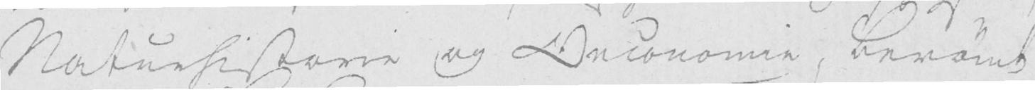Naturhistorie og Oeconomie, berømt
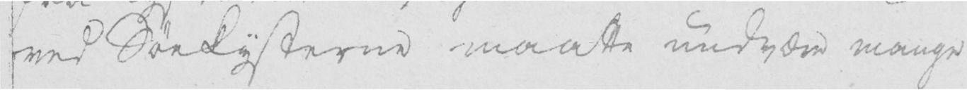ved Søekysterne maatte undvære mange
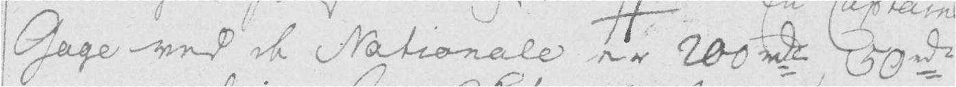Gage ved de Nationale er 200 rd, 50 rd
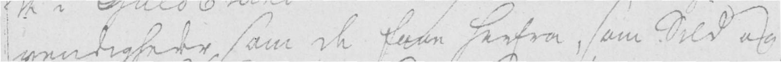vendigheder som de faae herfra, som Sild og
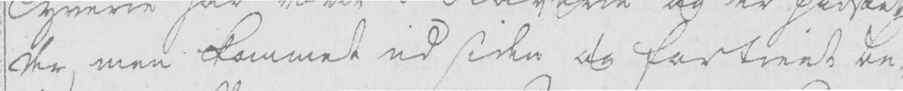der, men kommet ud siden og fortient be-
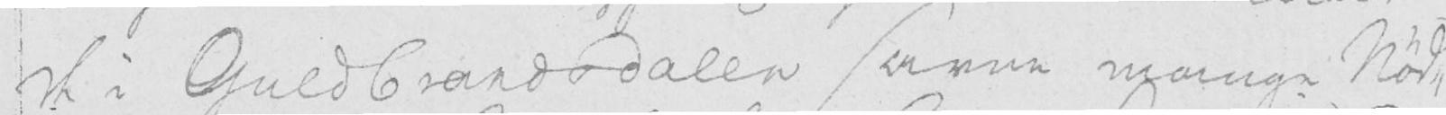de i Guldbrandsdalen savne mange Nød-
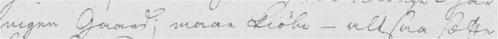ingen Gaard; maae kiøbe - altsaa sætte
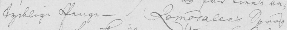tydelige Penge. Romsdalens Sprog
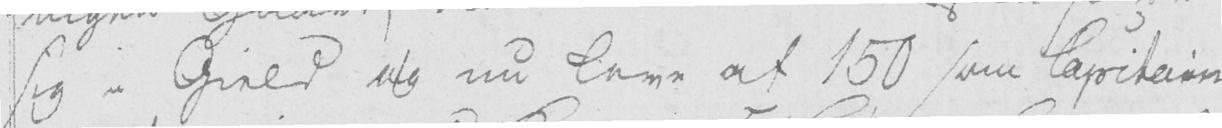sig i Gield og nu leve af 150 som Capitain
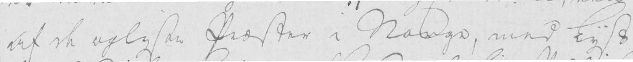af de oplyste Præster i Norge, med Lyst
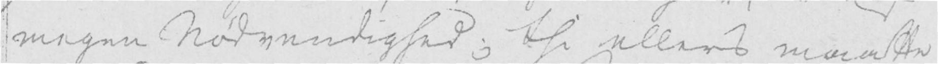megen Nødvendighed; thi ellers maatte
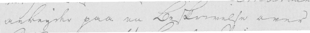arbeider paa en Beskrivelse over
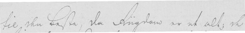til den beste, da Riigdom er et alt; de
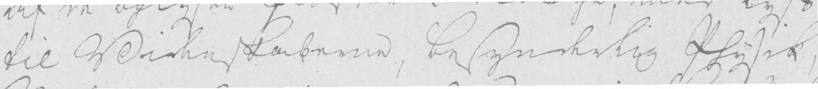til Videnskaberne, besynderlig Physik,
